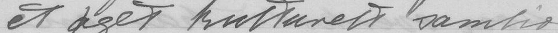et øget kulturelt samled
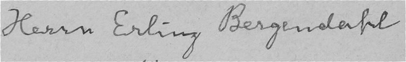Herrn Erling Bergendahl
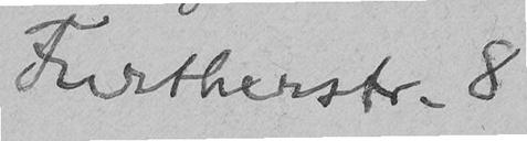Furtherstr. 8
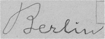Berlin
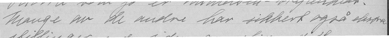Mange av de andre har sikkert også ekstra-
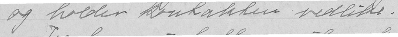og holder kontakten vedlike.
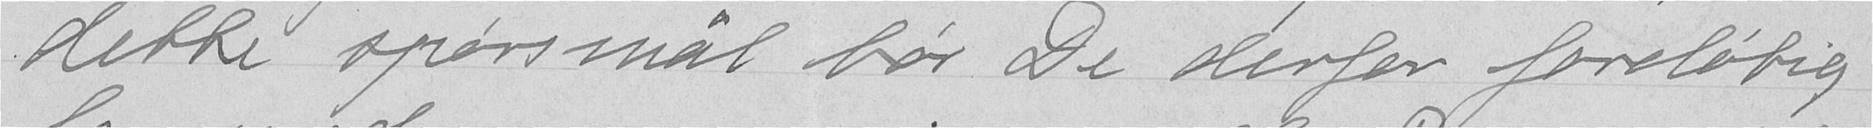dette spørsmål bør De derfor foreløbig
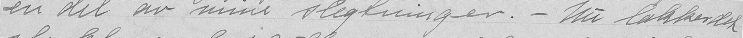en del av mine slegtninger. - Nu lakker det
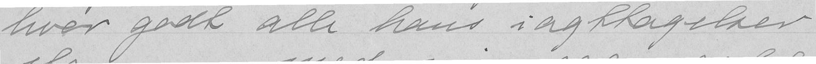hvor godt alle hans iagttagelser
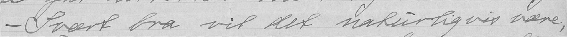- Svært bra vil det naturligvis være,
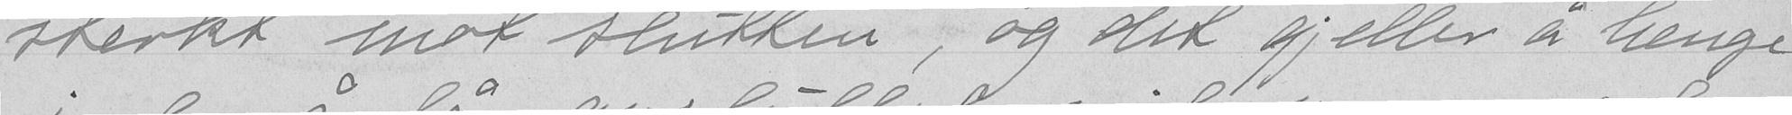sterkt mot slutten; og det gjeller å henge
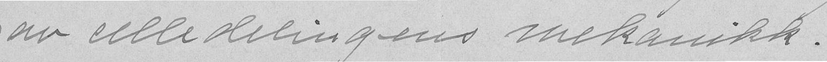av celledelingens mekanikk.
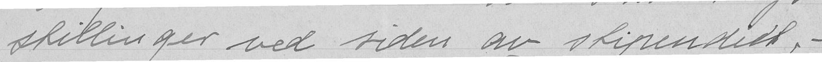stillinger ved siden av stipendiet.-
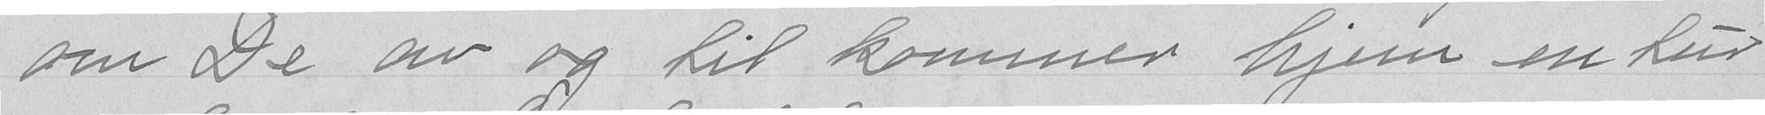om De av og til kommer hjem en tur
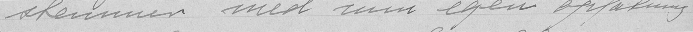stemmer med min egen opfatning
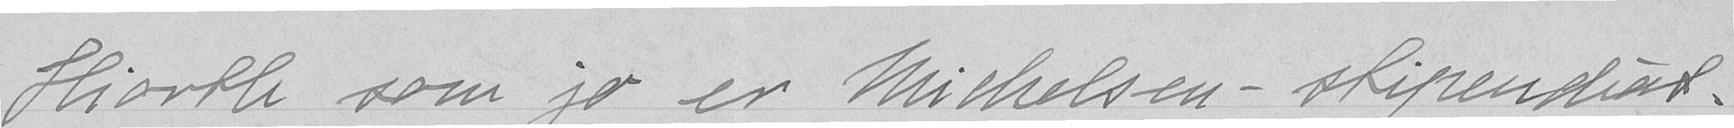Hjorth som jo er Michelsen-stipendiat.
